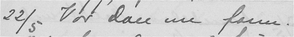22/5 Var Don om form.
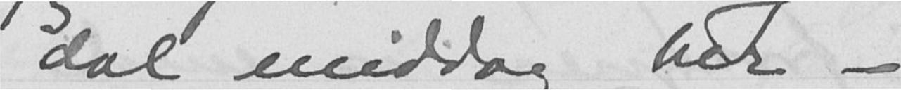dal middag her -
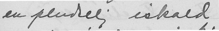en pludselig iskald
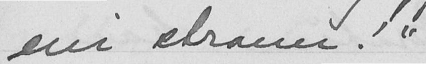eni stram!"
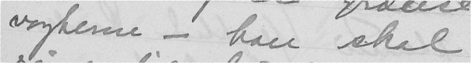vogterne - han skal
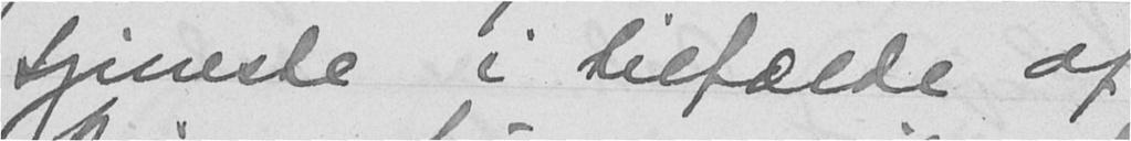tjeneste i tilfælde af
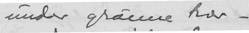under grønne trær -
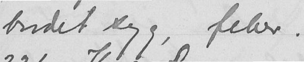bordet syg, feber.
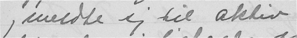og meldte sig til aktiv
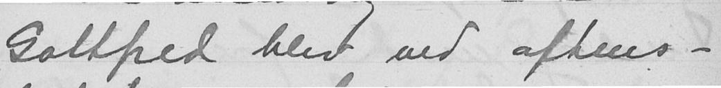Gottfred blev ved aftens-
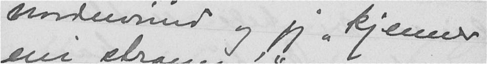nordenvind og jeg "kjenner
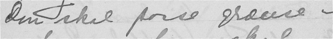Don skal passe grænse-
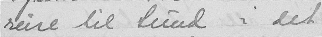reise til Sund i det
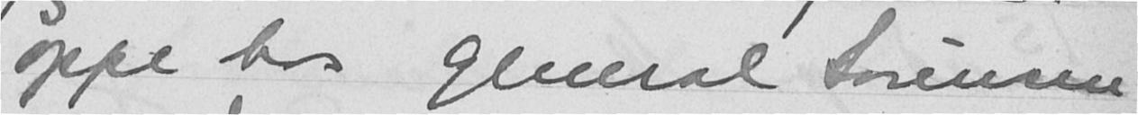oppe hos general Sørensen
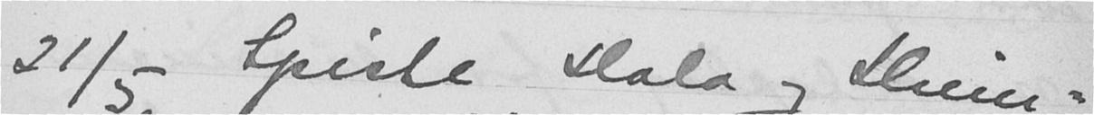21/5 Spiste Hola og Heim-
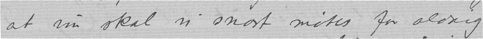at nu skal vi snart møtes for aldrig
